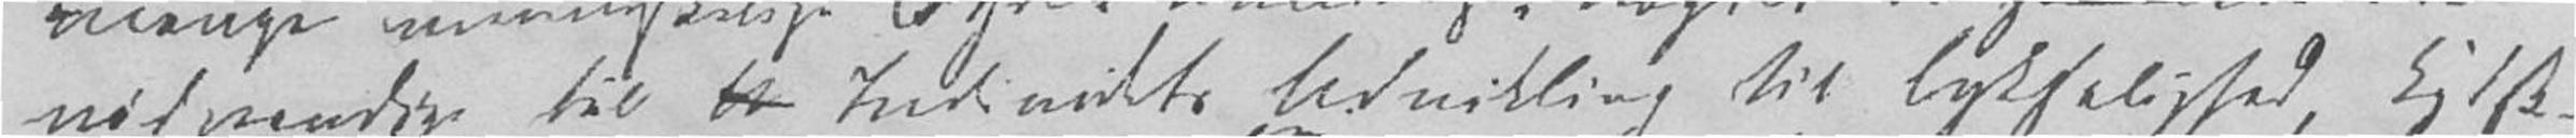nødvendige til Individets Udvikling til Lyksalighed, Kysk-
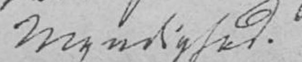Myndighed.
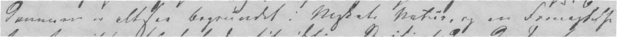dommen er altsaa begrundet i Mskets Natur, og en Fornægtelse
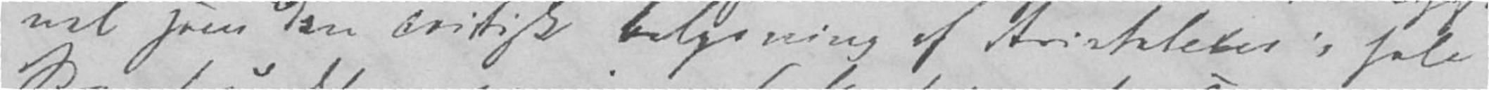vel som den critisk Belysning af Aristoteles's hele
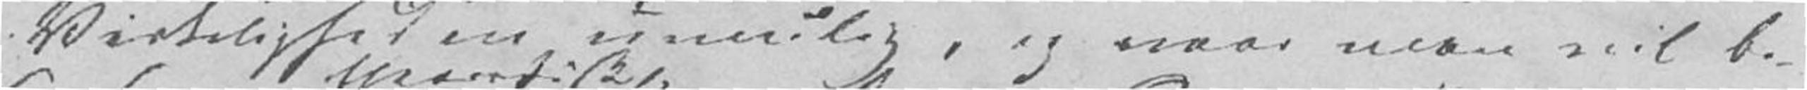Virkeligheden umulig, og naar man vil be-
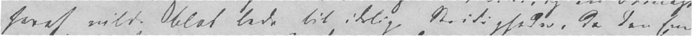heraf vilde blot lede til idelige Stridigheder, da den Ene
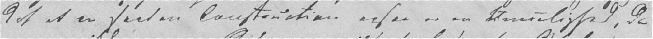det at en saadan Construction ogsaa er en Umulighed, da
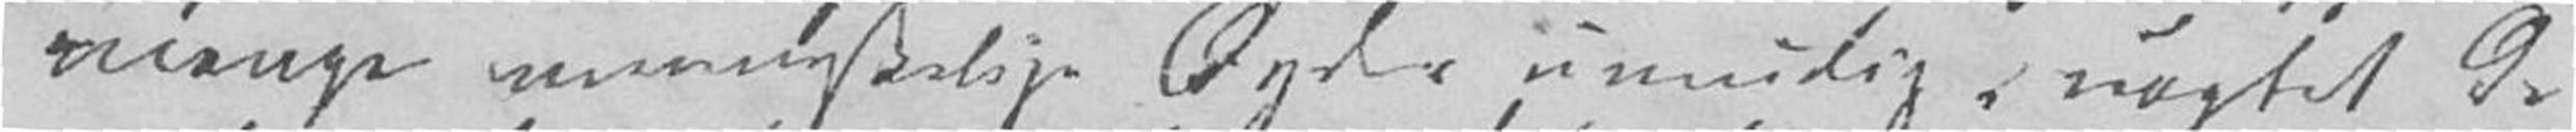mange menneskelige Dyder umulig, uagtet de
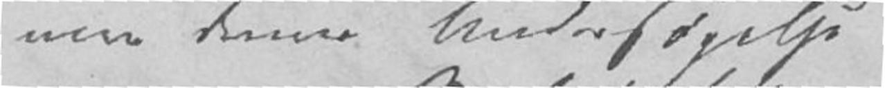men denne Undersøgelse
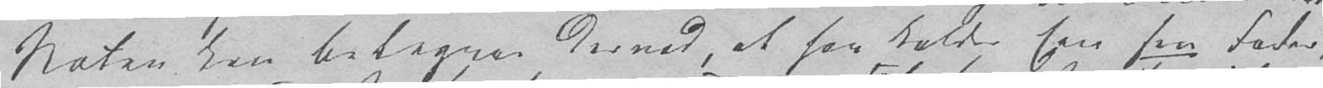Staten kan betegnes derved, at han kalder Een sin Fader,
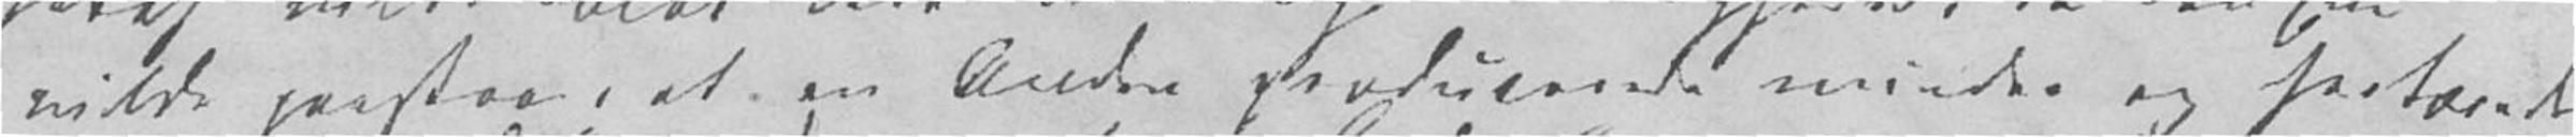vilde paastaa, at en Anden producerede mindre og fortærede
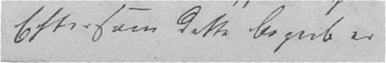Eftersom dette Begreb er
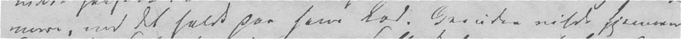mere, end det faldt paa hans Lod. Desuden vilde gjennem
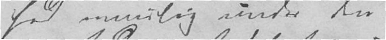hed umulig under den
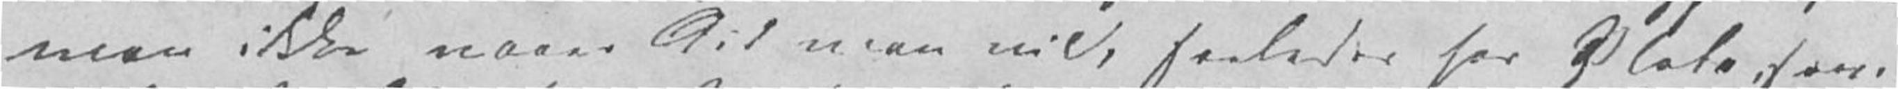man ikke naaer did man vil, saaledes for Plato, som
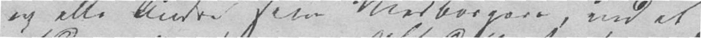og alle Andre sine Medborgere, ved at
